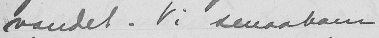vandet. Vi smaabarn
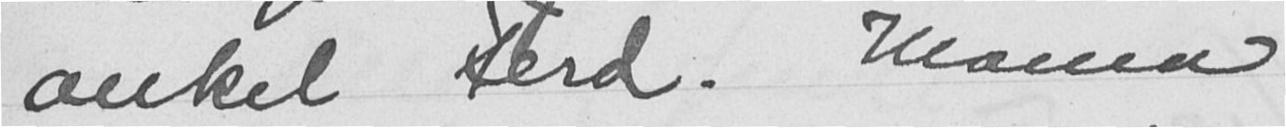onkel Ferd. Mama
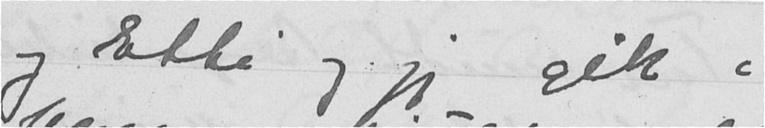og Etti og jeg gik i
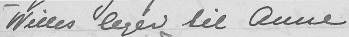Willes leger til Anne
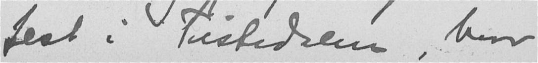fest i Tistedalen, hvor
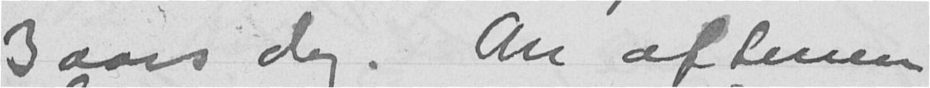3 aars dag. Om aftenen
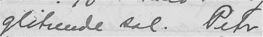glitrende sol. Peter,
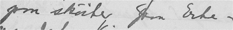paa skøiter paa Erte-
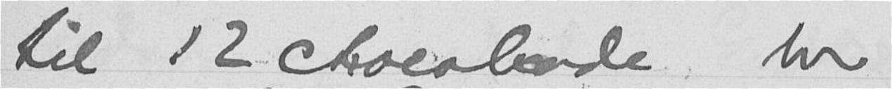til 12 chocolade her
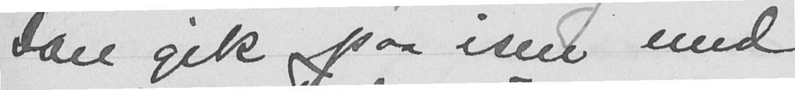Don gik paa isen med
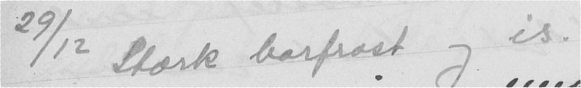29/12 Stærk barfrost og is.
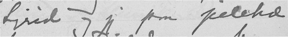Sigrid og jeg paa juletræ
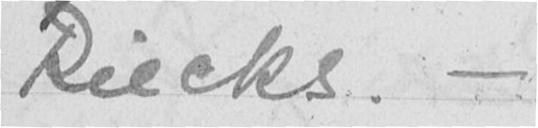Riecks. -
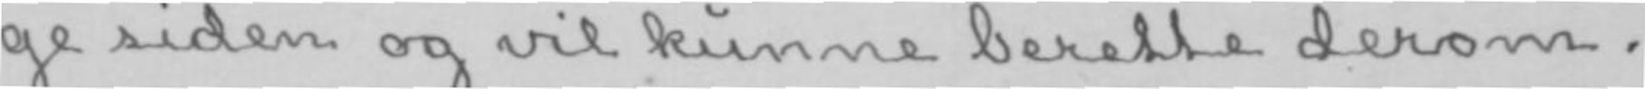ge siden og vil kunne berette derom.
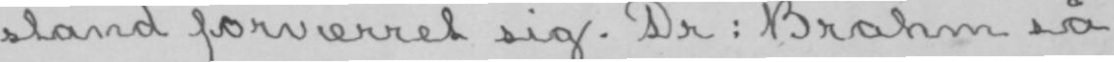stand forværret sig. Dr: Brahm så
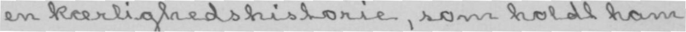en kærlighedshistorie, som holdt ham
